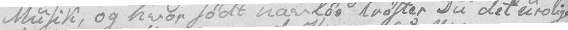Musik, og hvor sødt navløs trøster Du det urolige
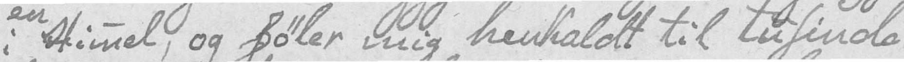i en Himmel, og føler mig henkaldt til tusinde
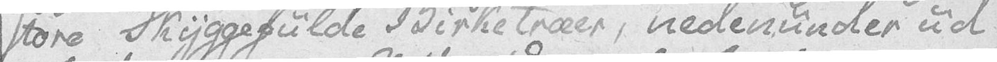store Skyggefulde Birketræer, nedenunder ud
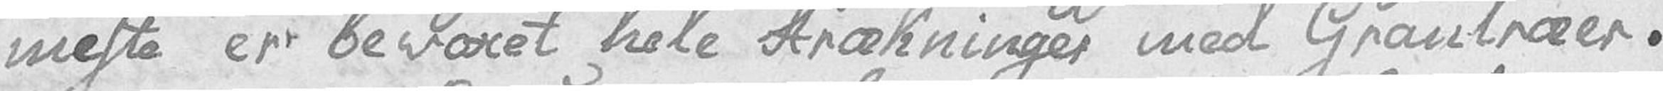meste er bevoxet hele Strækninger med Grantræer.
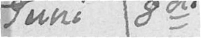Juni 8de
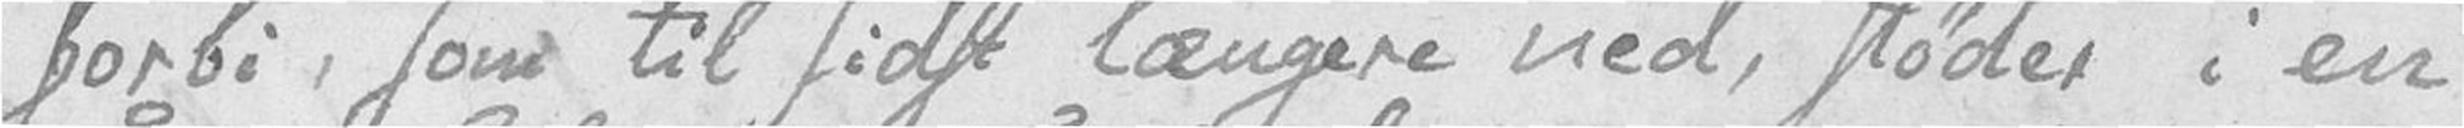forbi, som til sidst længere ned, støder i en
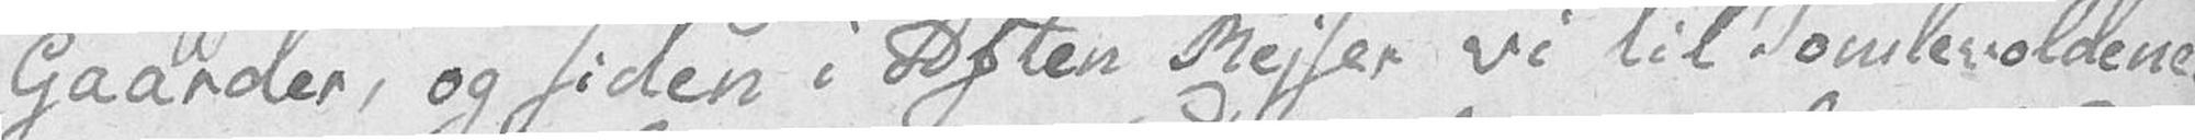Gaarder, og siden i Aften Rejser vi til Tomlevoldene.
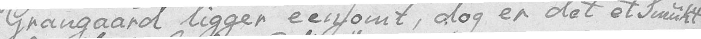Grangaard ligger eensomt, dog er det et Smukt
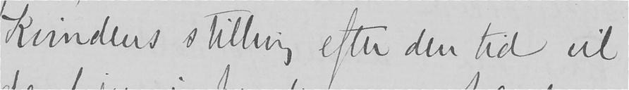Kvindens stilling efter den tid vil
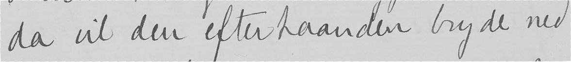da vil den efterhaanden bryde ned
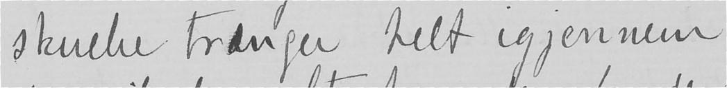skuelse trænger helt igjennem
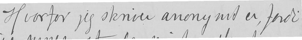Hvorfor jeg skriver anonymt er, fordi
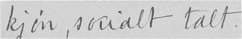kjøn, socialt talt.
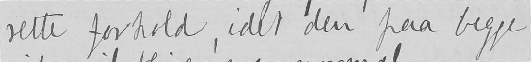rette forhold, idet den paa begge
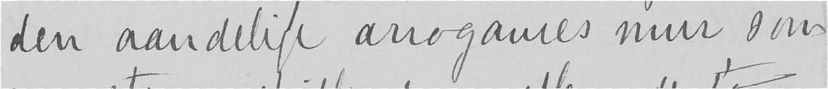den aandelige arrogances mur som
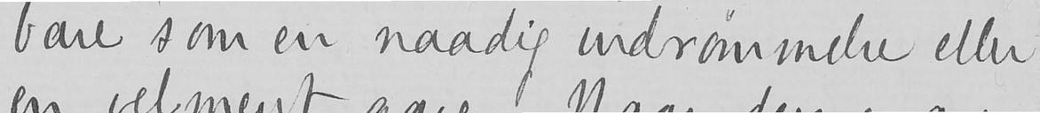bare som en naadig indrømmelse eller
